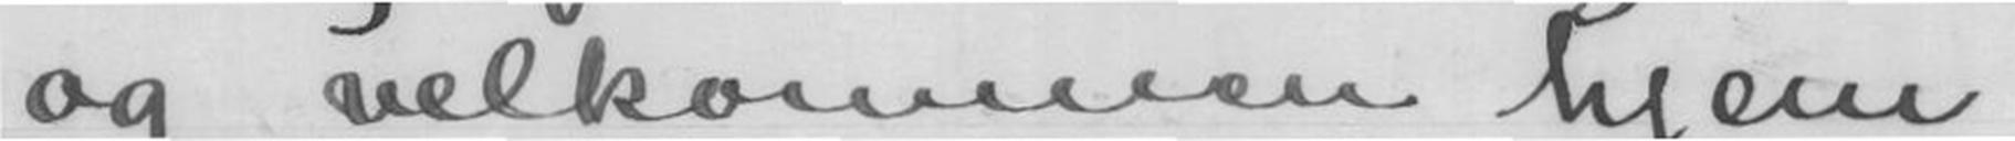og velkommen hjem
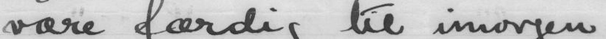være færdig til imorgen,
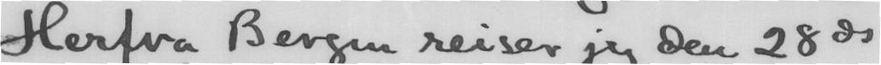Herfra Bergen reiser jeg den 28de
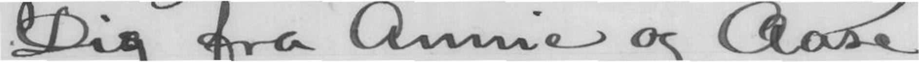Dig fra Annie og Aase
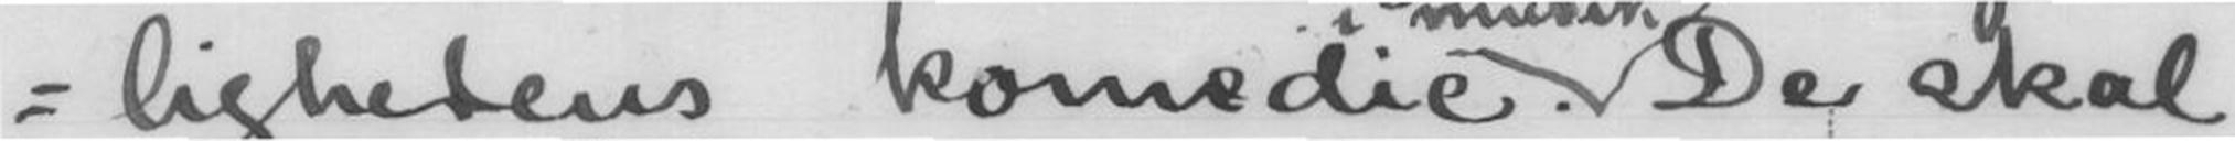lighedens komedie. De skal
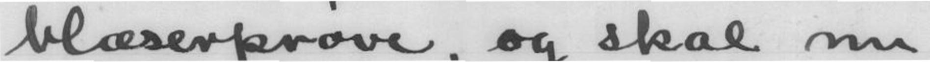blæserprøve, og skal nu
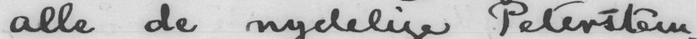alle de nydelige Peterstem-
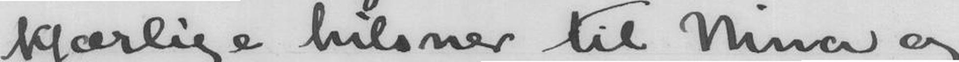kjærlige hilsner til Nina og
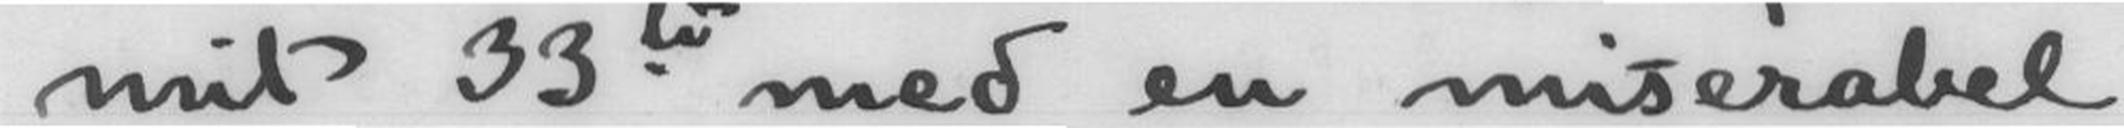mit 33te, med en miserabel
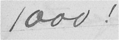1000!
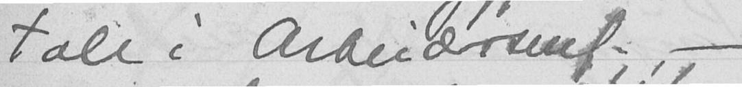tale i Arbeidersmf. -
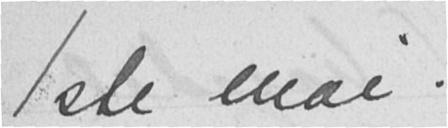1ste mai.
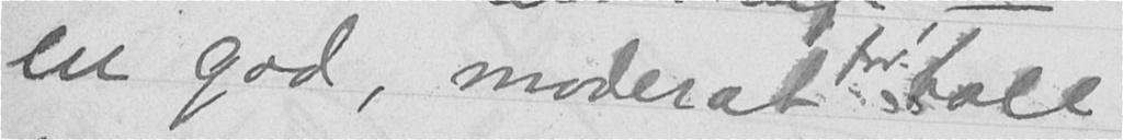en god, moderat tale
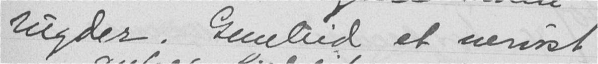rugder. Gunhild et nervøst
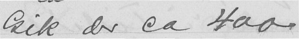Gik der ca 400
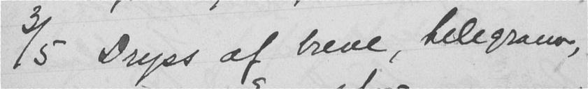3/5 Dryss af breve, telegram,
